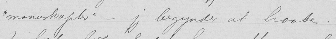"manuskripter" - jeg begynder at haabe.
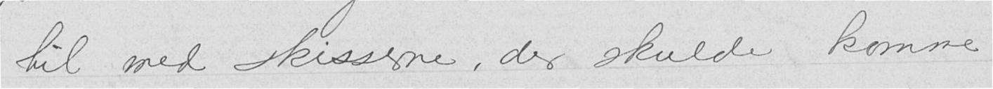til med skisserne, der skulde komme
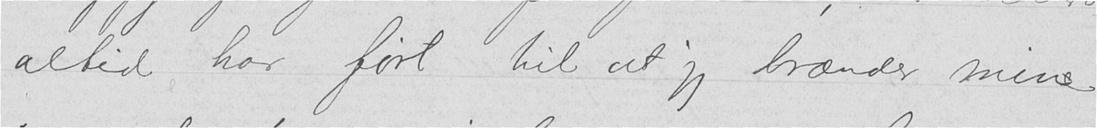altid har ført til at jeg brænder mine
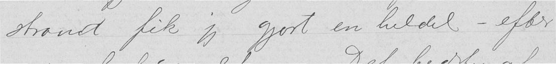strand fik jeg gjort en heldel - efter
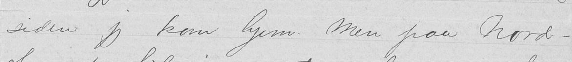siden jeg kom hjem. Men paa Nord-
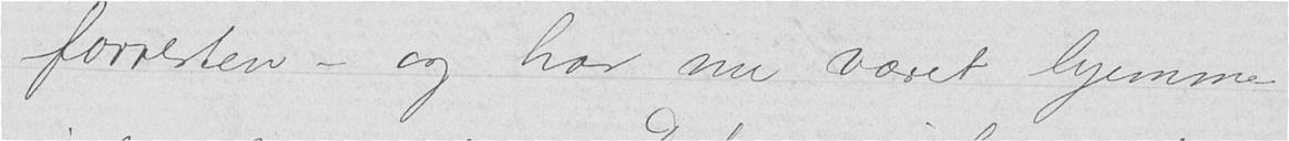forresten - og har nu været hjemme
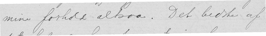mine forhold altsaa. Det bedste af
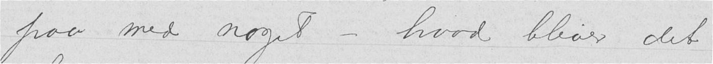paa med noget - hvad bliver det
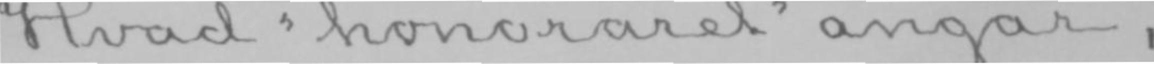Hvad «honoraret» angår,
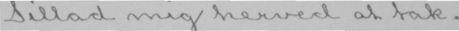Tillad mig herved at tak-
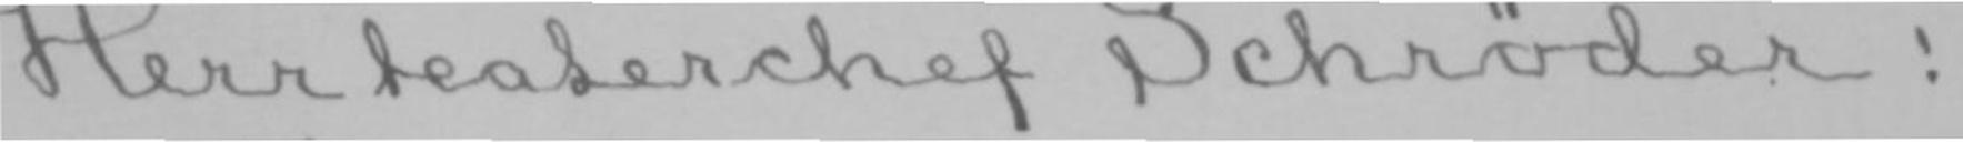Herr teaterchef Schrøder!
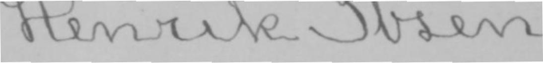Henrik Ibsen.
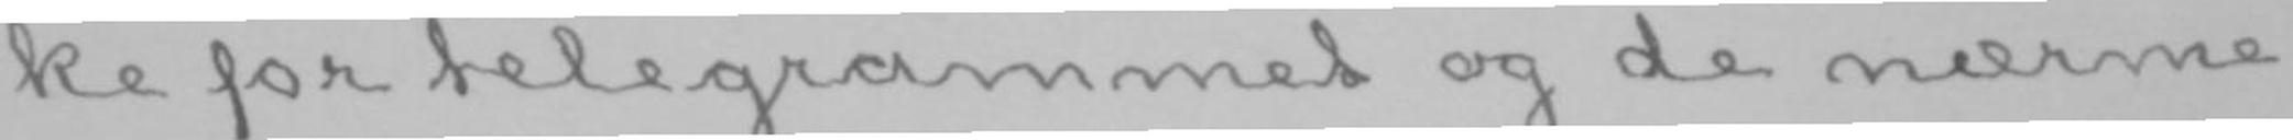ke for telegrammet og de nærme-
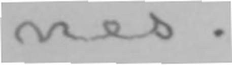nes.
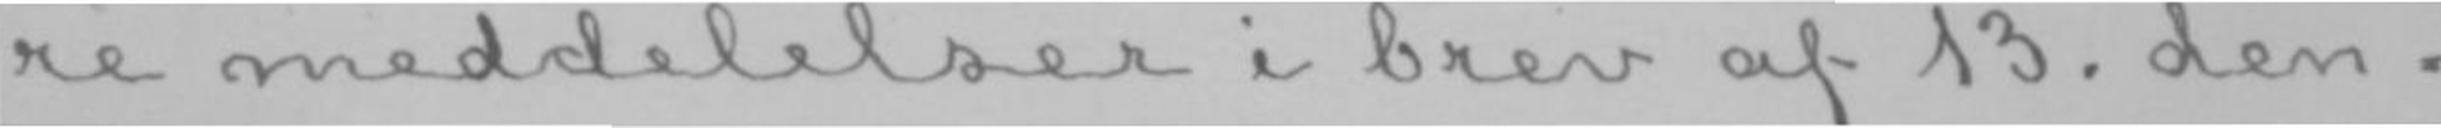re meddelelser i brev af 13. den-
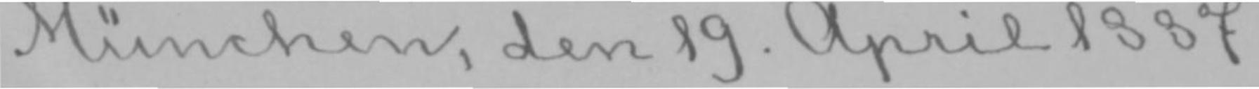München, den 19. April 1887.
